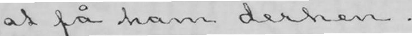at få ham derhen.
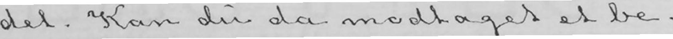det. Kan du da modtaget et be-
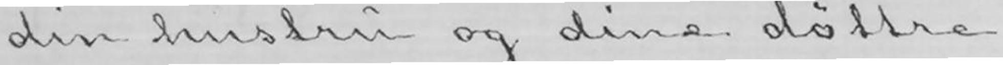din hustru og dine døttre.
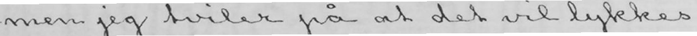men jeg tviler på at det vil lykkes
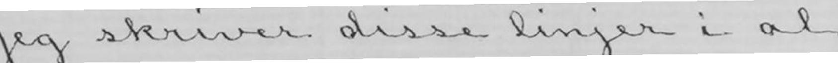Jeg skriver disse linjer i al
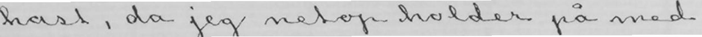hast, da jeg netop holder på med
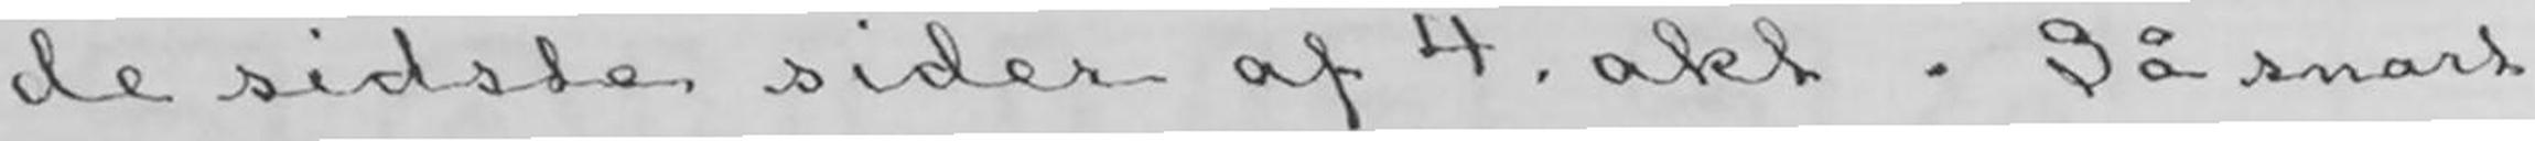de sidste sider af 4. akt. Så snart
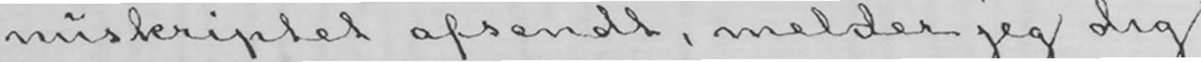nuskriptet afsendt, melder jeg dig
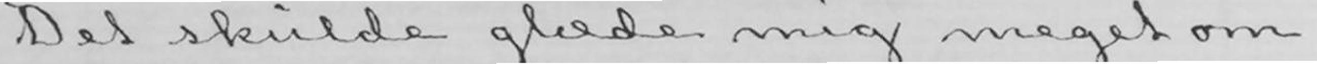Det skulde glæde mig meget om
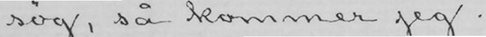søg, så kommer jeg.
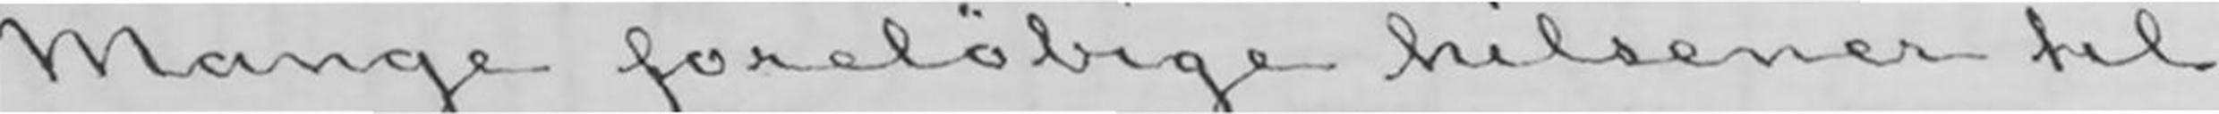Mange foreløbige hilsener til
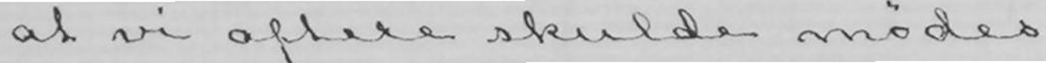at vi oftere skulde mødes.
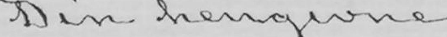Din hengivne
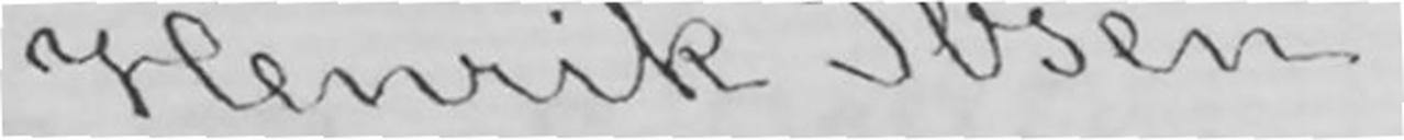Henrik Ibsen.
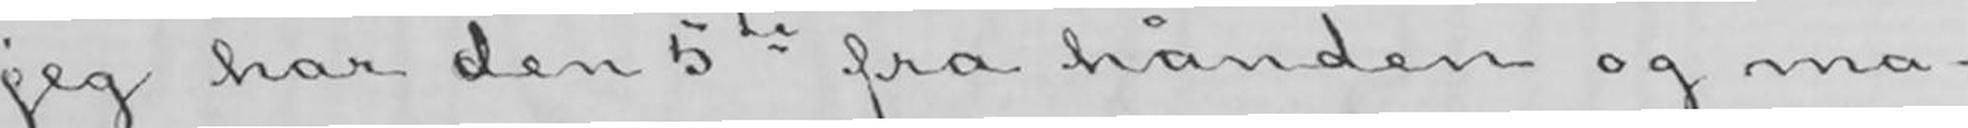jeg har den 5te fra hånden og ma-
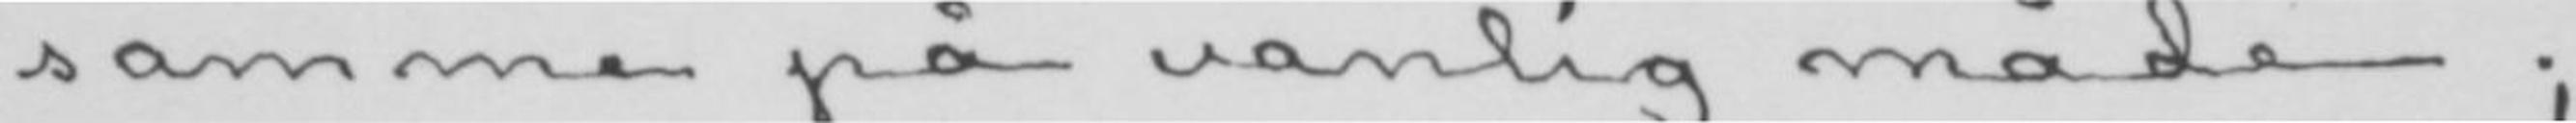samme på vanlig måde;
